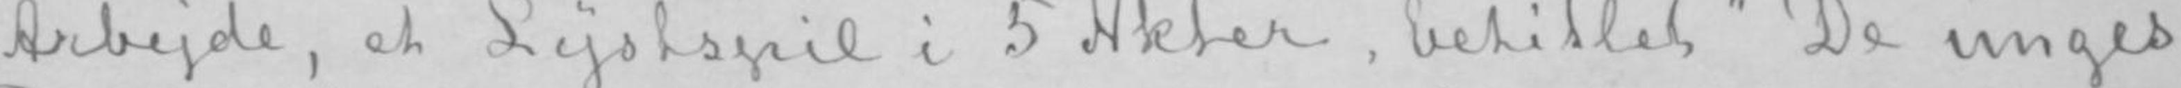Arbejde, et Lystspil i 5 Akter, betitlet «De unges
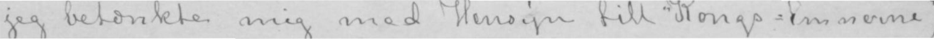jeg betænkte mig med Hensyn till «Kongs-Emnerne»,
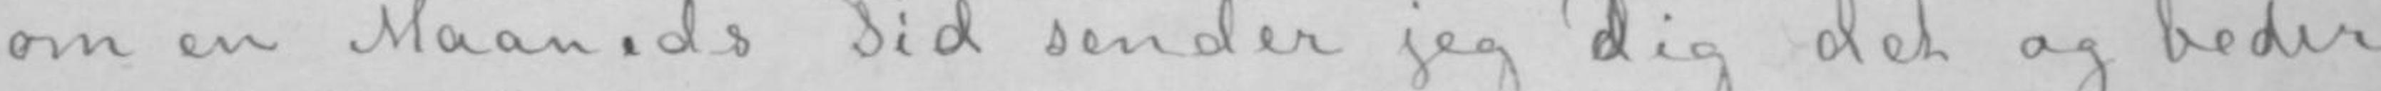om en Maaneds Tid sender jeg Digdig det og beder
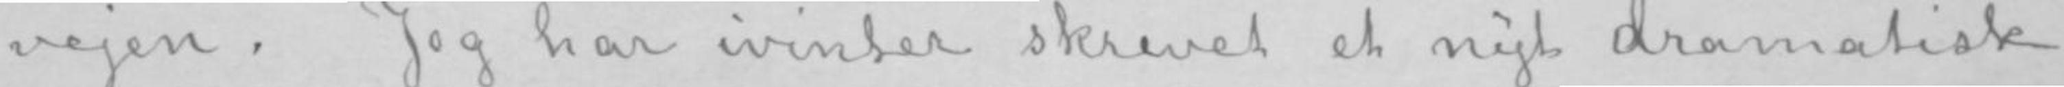vejen. Jeg har ivinter skrevet et nyt dramatisk
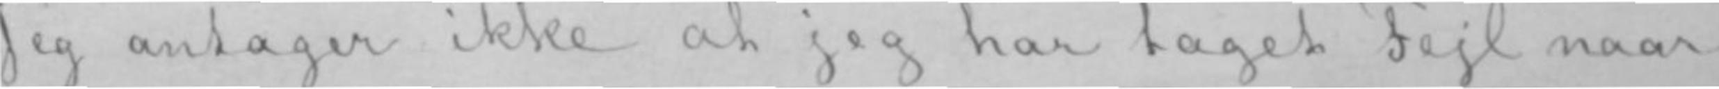Jeg antager ikke at jeg har taget Fejl naar
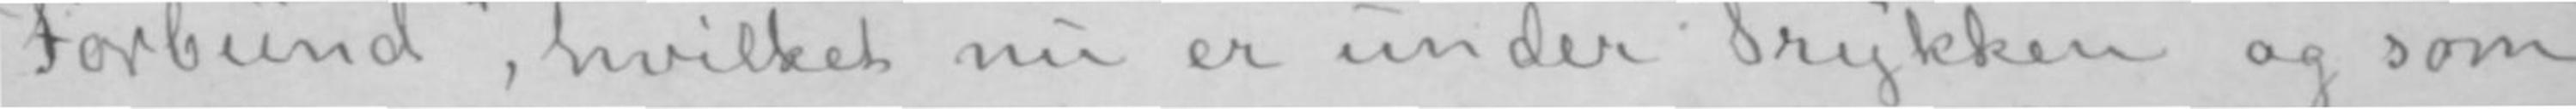Forbund», hvilket nu er under Trykken og som
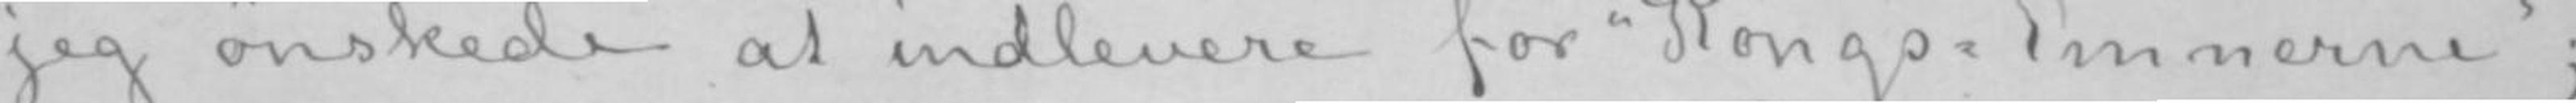jeg ønskede at indlevere før «Kongs-Emnerne»;
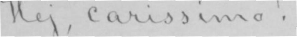Hej, carissimo!
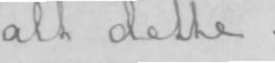alt dette.
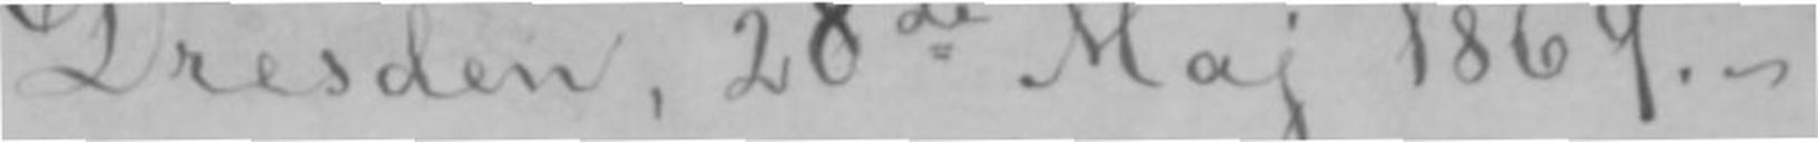Dresden, 28de Maj 1869.-
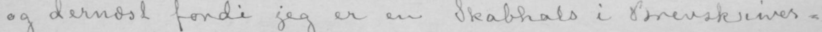og dernæst fordi jeg er en Skabhals i Brevskriver-
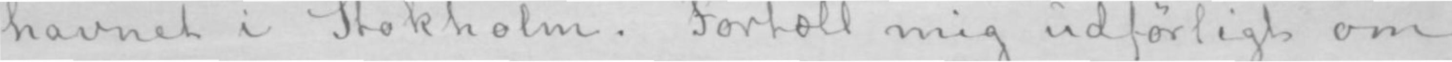havnet i Stokholm. Fortæll mig udførligt om
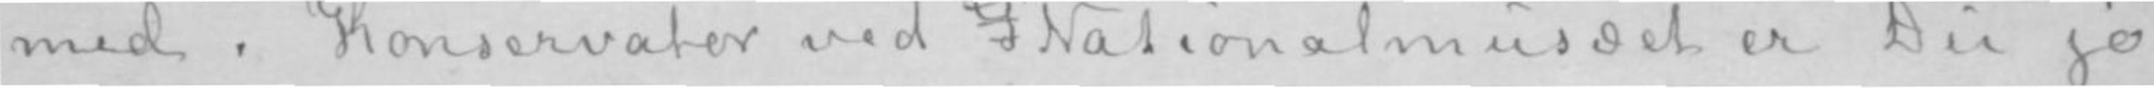med. Konservator ved  Nationalmusæet er Du jo
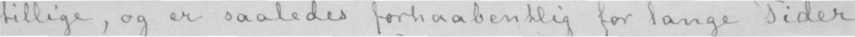tillige, og er saaledes forhaabentlig for lange Tider
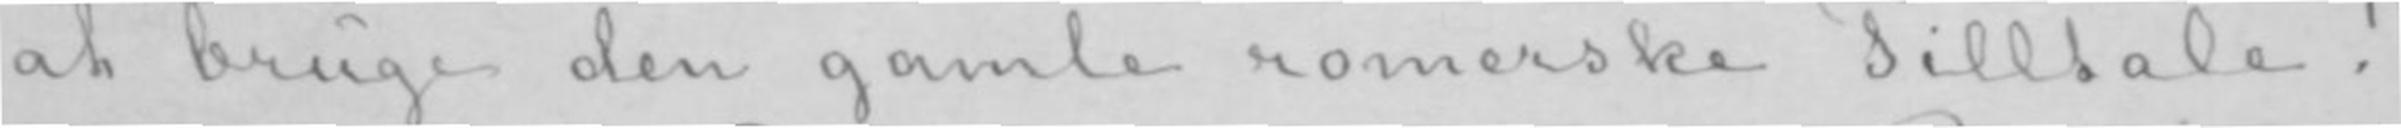at bruge den gamle romerske Tilltale!
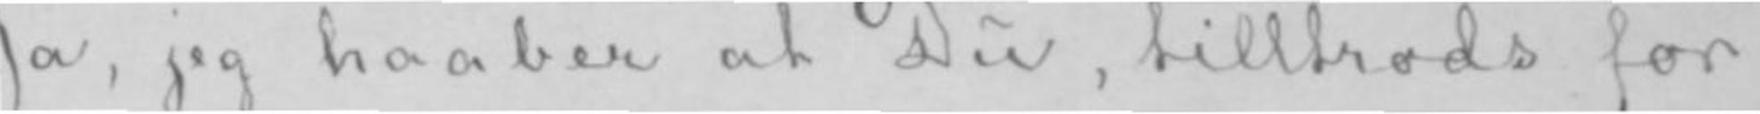Ja, jeg haaber at Du, tilltrods for
